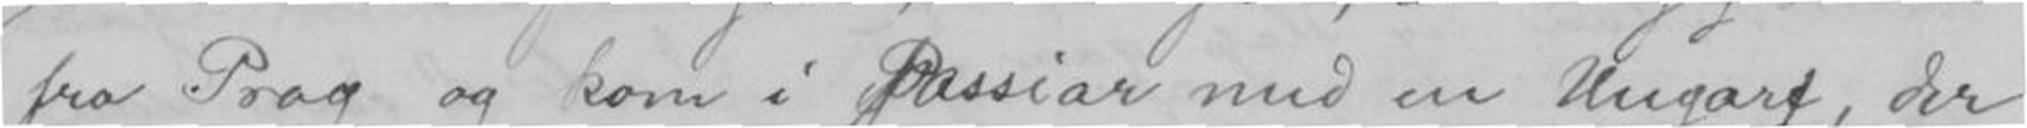fra Prag og kom i Passiar med en Ungar, der
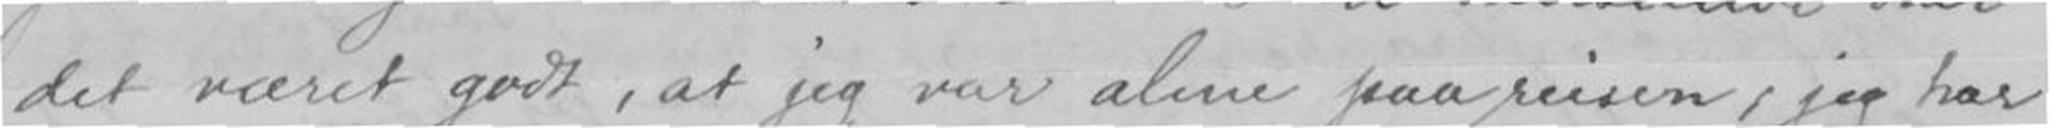det været godt, at jeg var alene paa reisen, jeg har
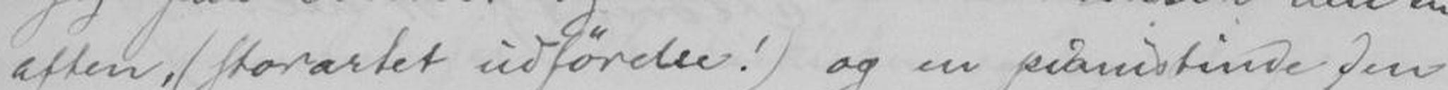aften, (storartet udførelse!) og en pianistinde den
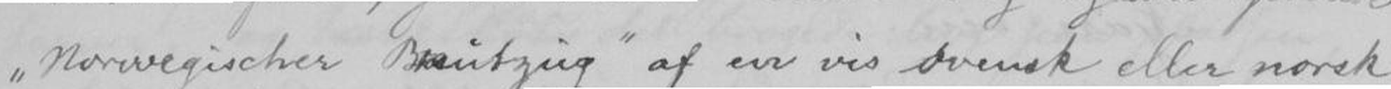"Norwegischer Bautzug?" af en vis svensk eller norsk
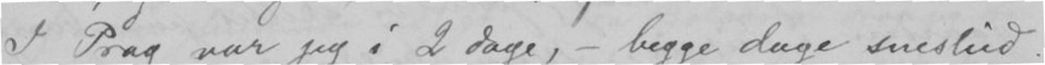I Prag var jeg i 2 dage, - begge dage sneslud.
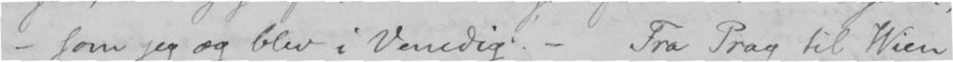- som jeg og blev i Venedig. - Fra Prag til Wien
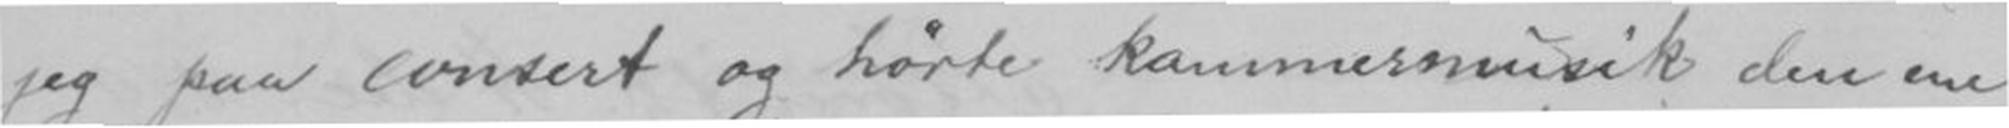jeg paa consert og hørte kammermusik den ene
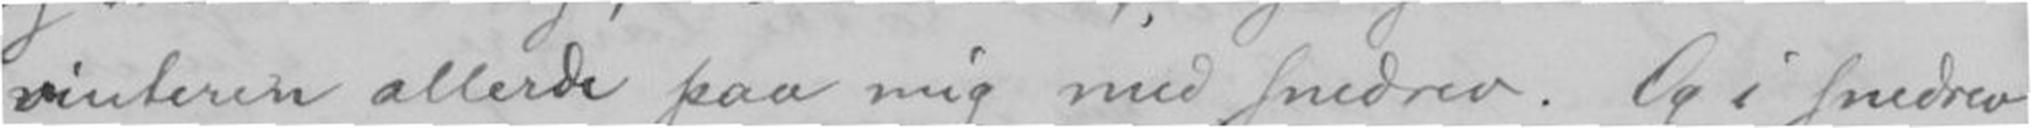vinteren allerede paa mig med snedrev. Og i snedrev
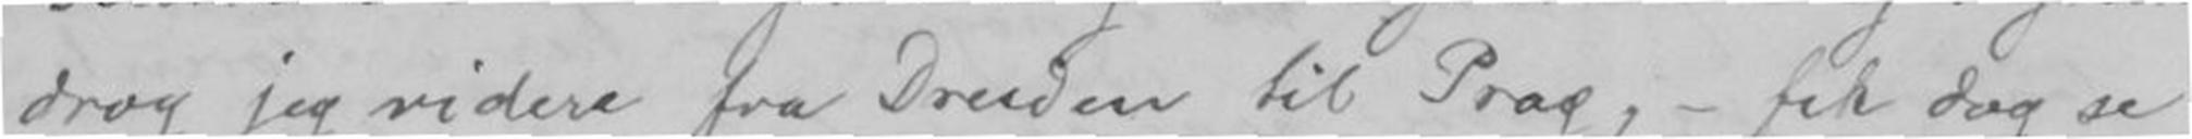drog jeg videre fra Dresden til Prag, - fik dog se
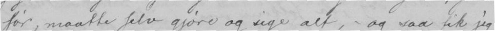før, maatte selv gjøre og sige alt, - og saa fik jeg
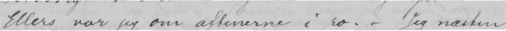Ellers var jeg om aftenerne i ro. - Jeg næsten
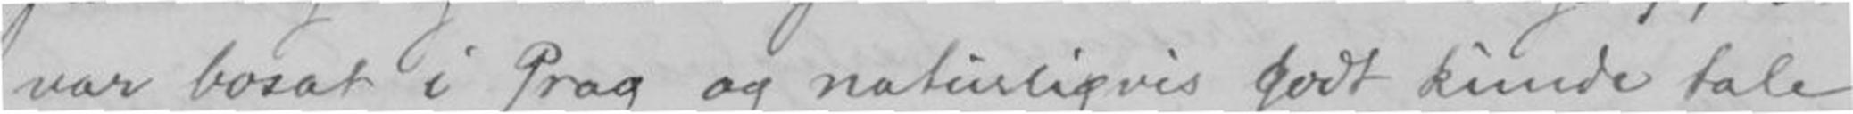var bosat i Prag og naturligvis godt kunde tale
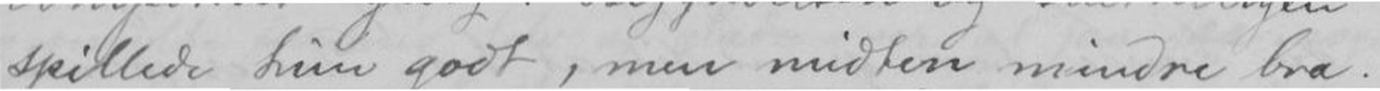spillede hun godt, men midten mindre bra.
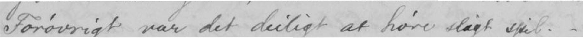Forøvrig var det deilig at høre sligt spil. -
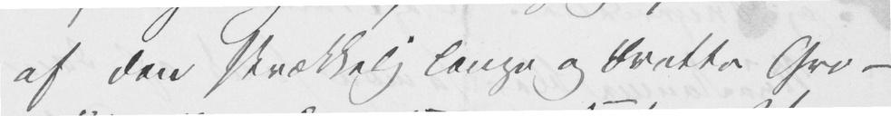af den skrækkelig lange og bratte Gro-
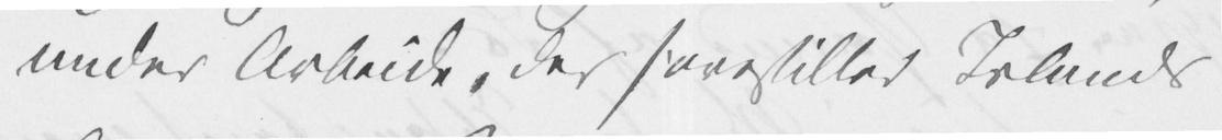under Arbeide, der forestiller Islands
